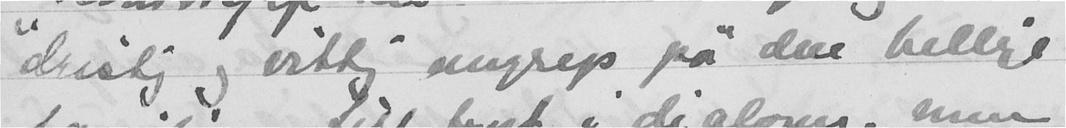dristig og vittig angrep på den hellige
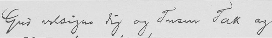Gud velsigne dig og Tusen Tak og
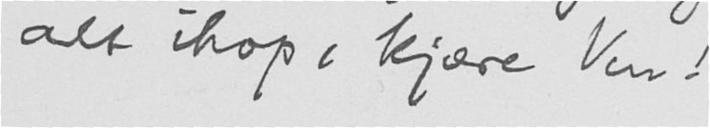alt ihop, kjære Ven!
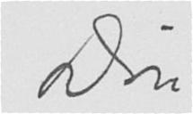Din
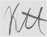KH
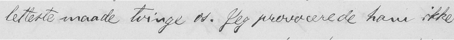letteste maade tvinge os. Jeg provocerede ham ikke
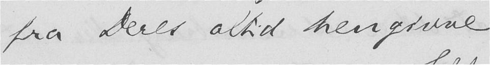fra Deres altid hengivne
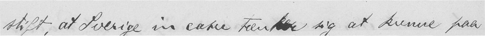stift, at Sverige in casu tænker sig at kunne paa
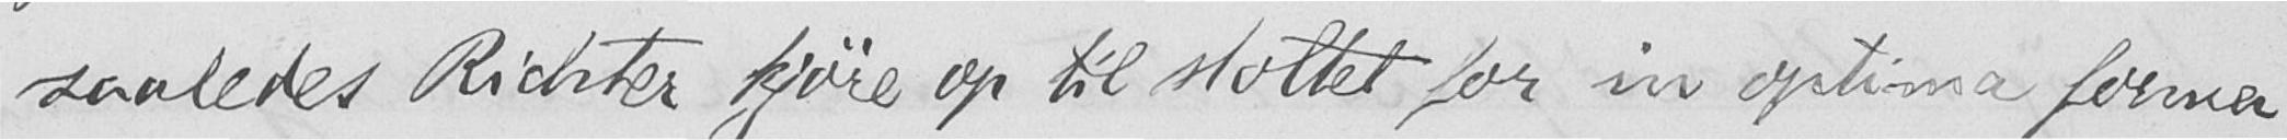saaledes Richter kjøre op til slottet for in optima forma
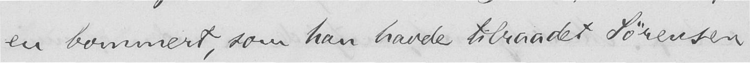en bommert, som han havde tilraadet Sørensen
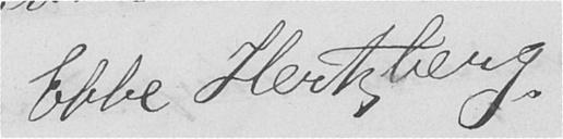Ebbe Hertzberg.
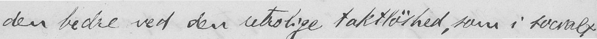den bedre ved den utrolige taktløshed, som i socialt
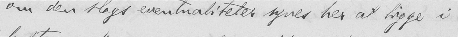om den slags eventualiteter synes her at ligge i
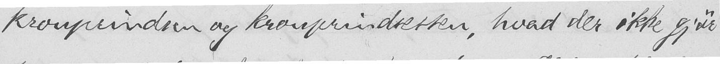kronprindsen og kronprindsessen, hvad der ikke gjør
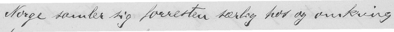Norge samler sig forresten særlig hos og omkring
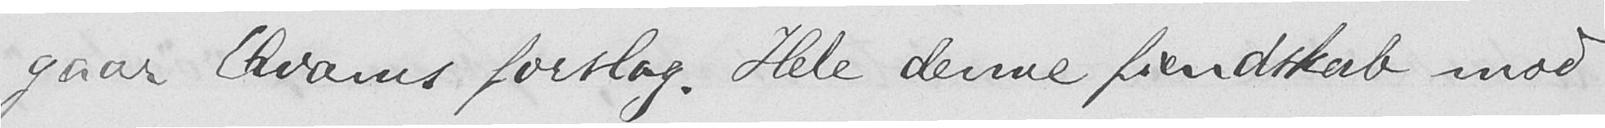gaar hvams forslag. Hele denne fiendskab mod
